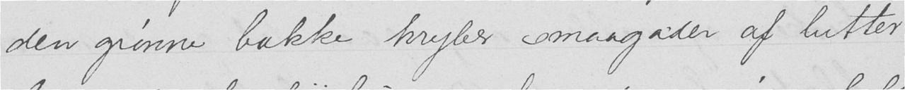den grønne bakke kryber smaagader af lutter
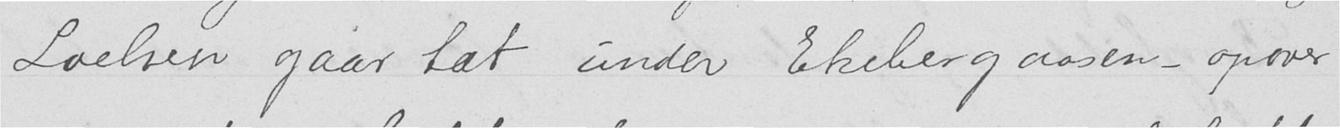Loelven gaar tæt under Ekebergaasen — opover
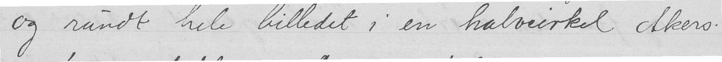og rundt hele billedet i en halvsirkel Akers.
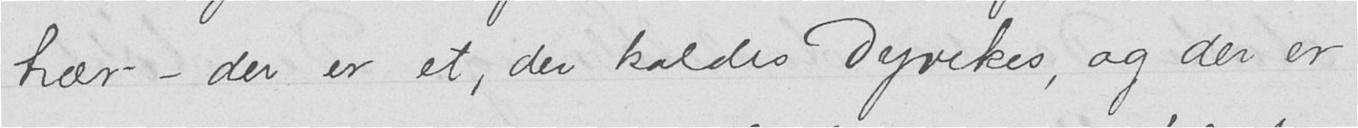trær — der er et, der kaldes Dyvekes, og der er
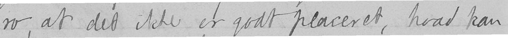ro, at det ikke er godt placeret, hvad kan
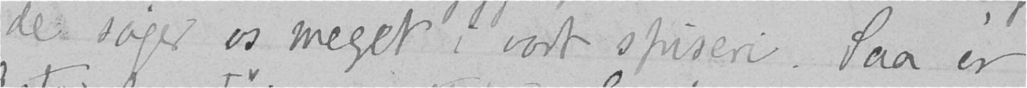de søger os meget i vort spiseri. Saa er
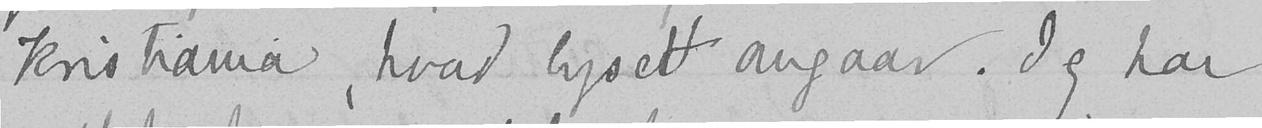Kristiania, hvad lyset angaar. Jeg har
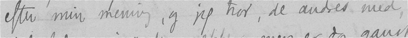efter min mening, og jeg tror, de andres med,
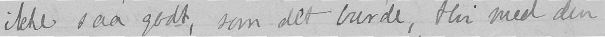ikke saa godt, som det burde, thi med den
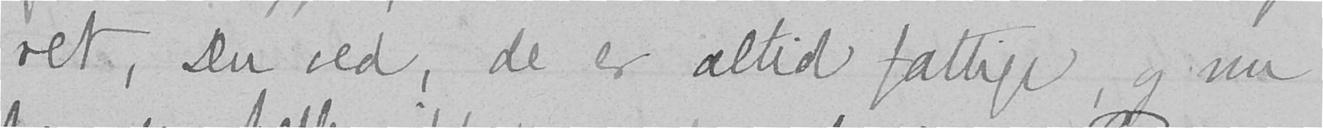ret, Du ved, de er altid fattige, og nu
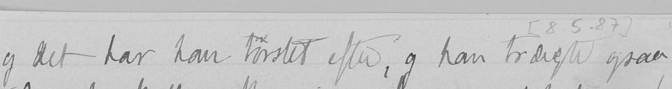og det har han tørstet efter, og han trængte ogsaa
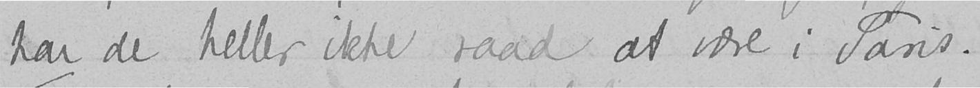har de heller ikke raad at være i Paris.
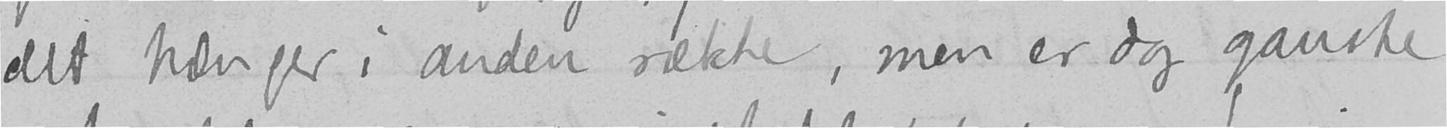det hænger i anden række, men er dog ganske
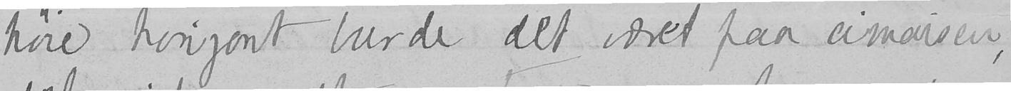høie horizont burde det været paa cimaisen,
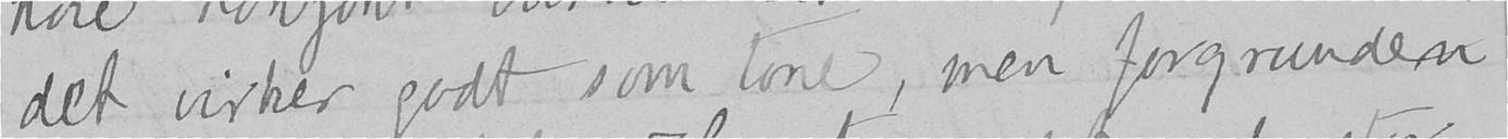det virker godt som tone, men forgrunden
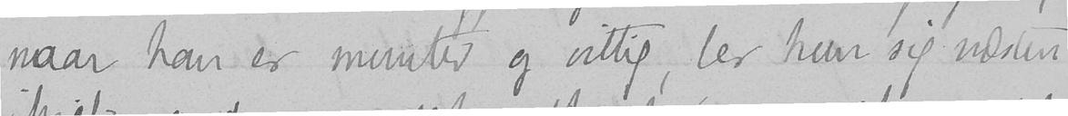naar han er munter og vittig, ler hun sig næsten
